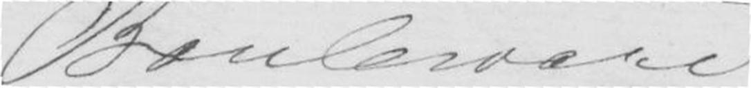Bouleward
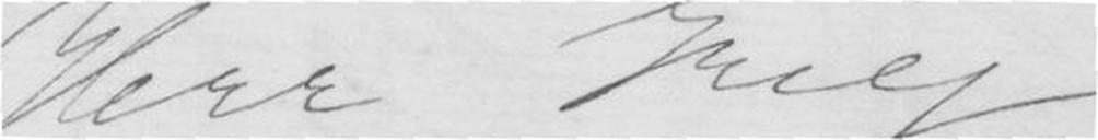Herr Grieg
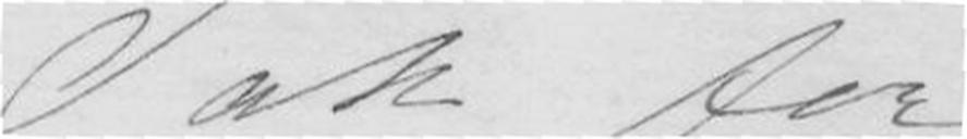Tak for
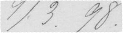9/3.98.
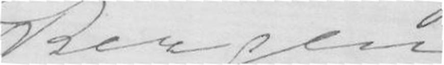i Bergen.
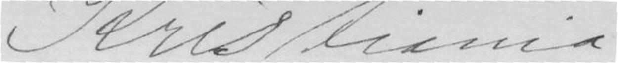Kristiania
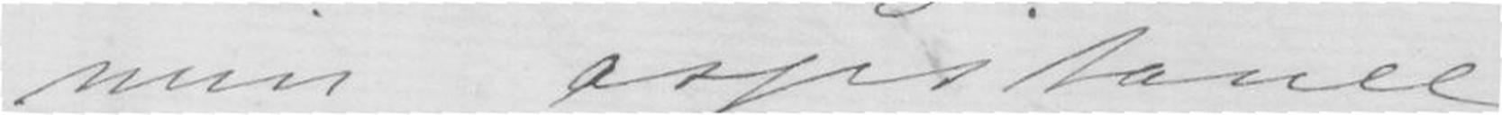min assistance
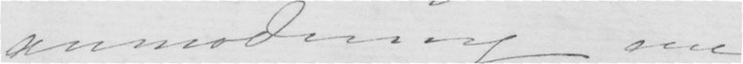anmodning om
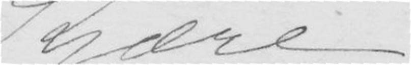Kjære
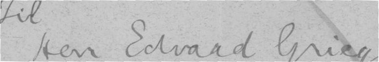Til Herr Edvard Grieg
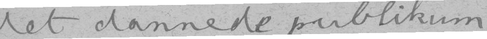det dannede publikum
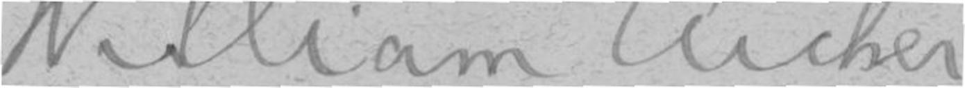William Archer
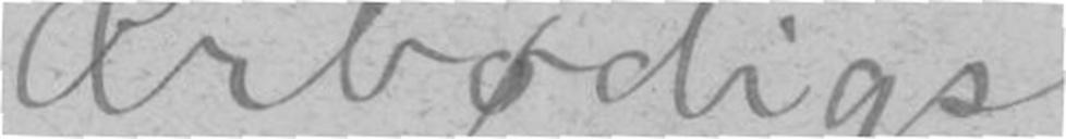Ærbødigst
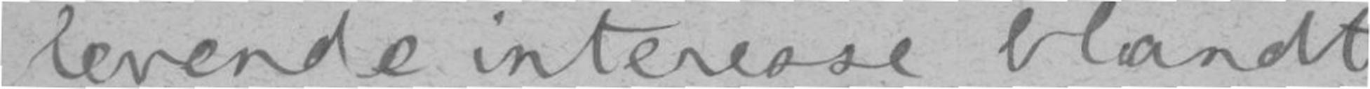levende interesse blandt
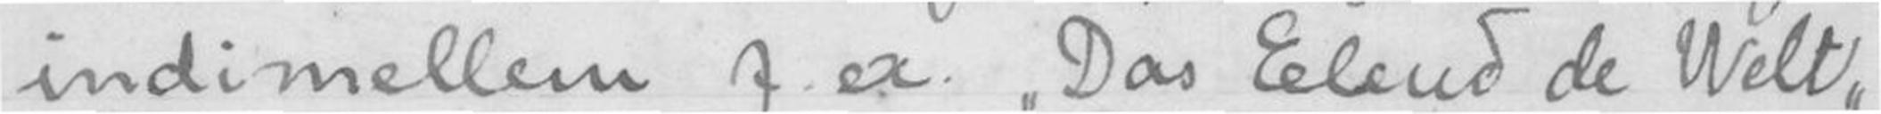indimellem f.ex. "Das Elend de Welt"
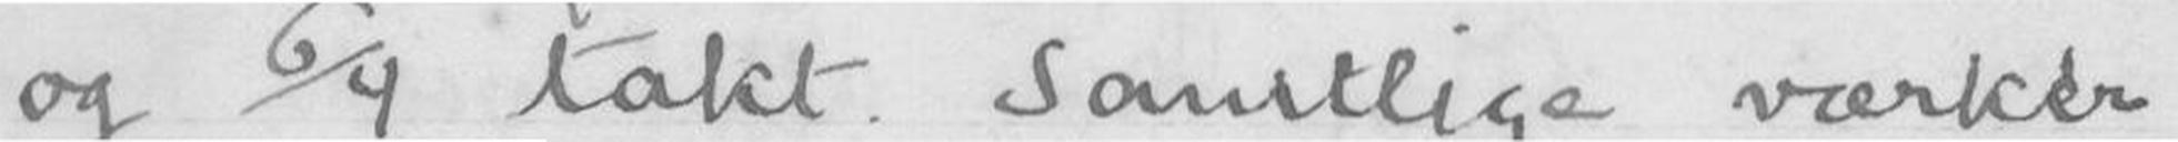og 6/4 takt. Samtlige værker
